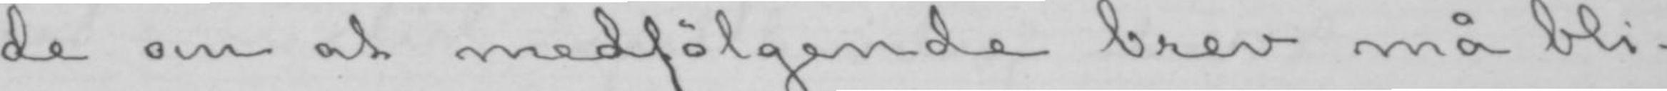de om at medfølgende brev må bli-
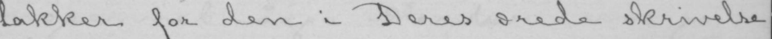takker for den i Deres ærede skrivelse
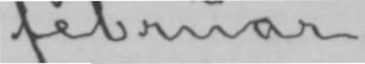februar
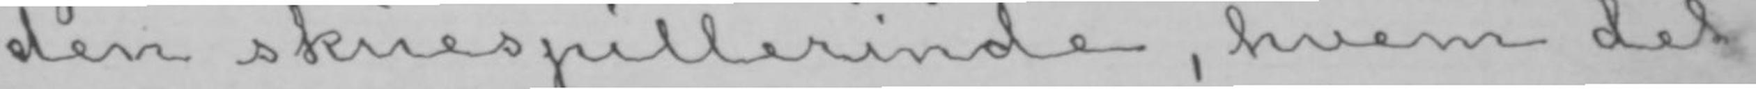den skuespillerinde, hvem det
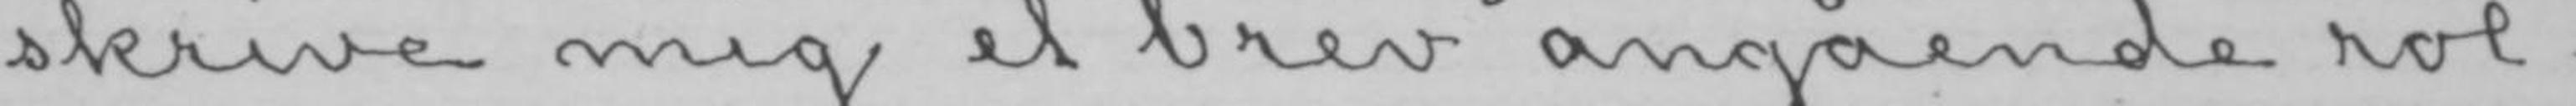skrive mig et brev angående rol-
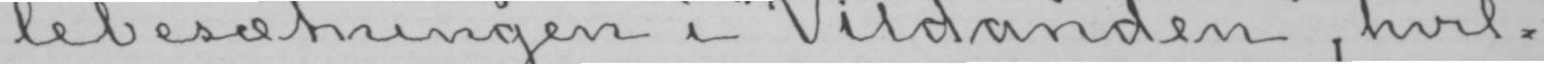lebesætningen i «Vildanden», hvil-
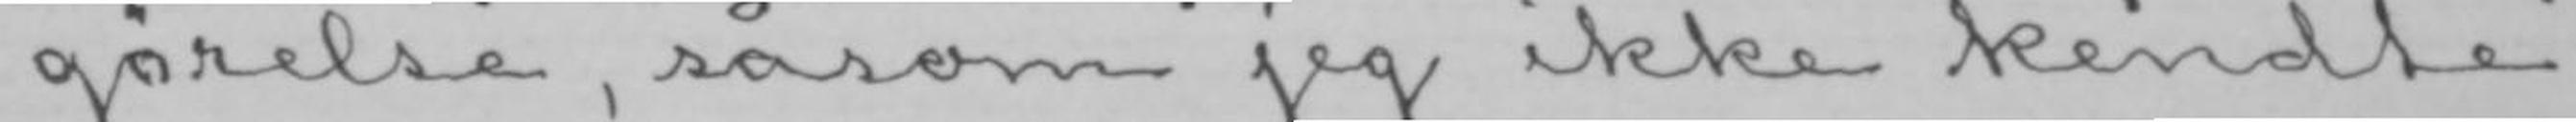gørelse, såsom jeg ikke kendte
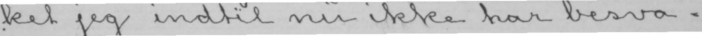ket jeg indtil nu ikke har besva-
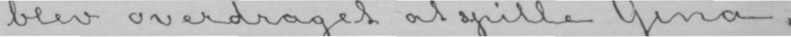blev overdraget at spille Gina,
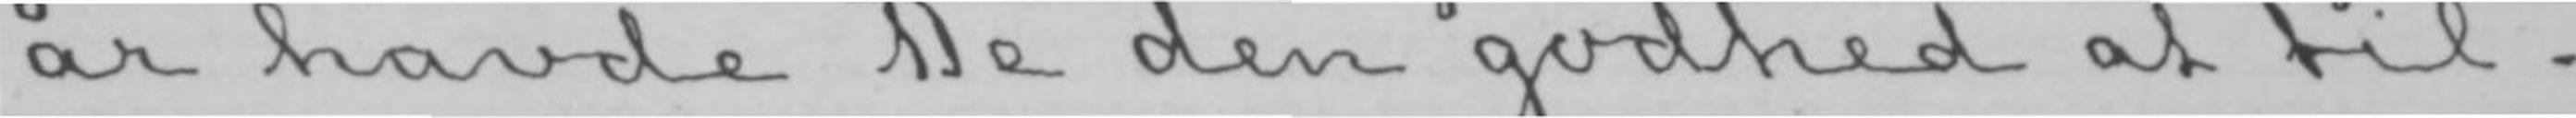år havde De den godhed at til-
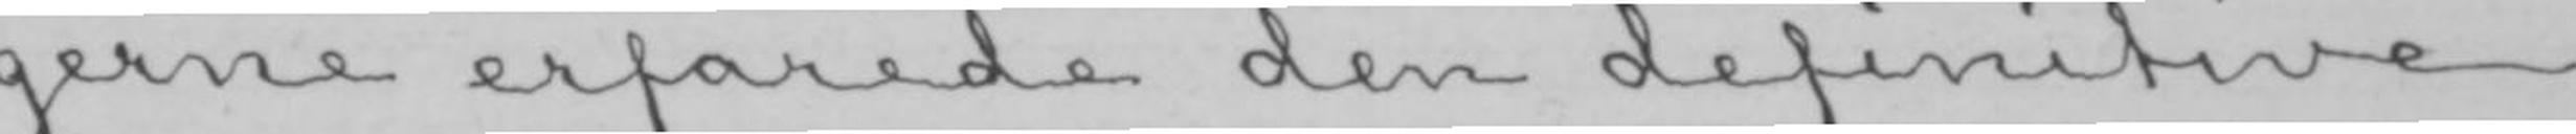gerne erfarede den definitive
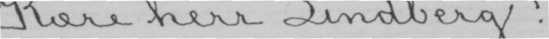Kære herr Lindberg!
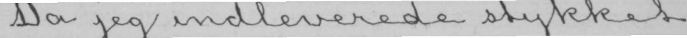Da jeg indleverede stykket
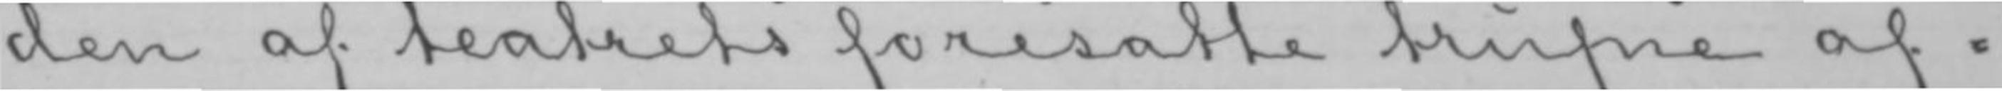den af teatrets foresatte trufne af-
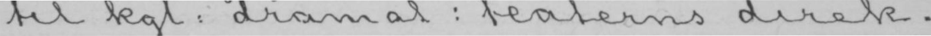til kgl: dramat: teaterns direk-
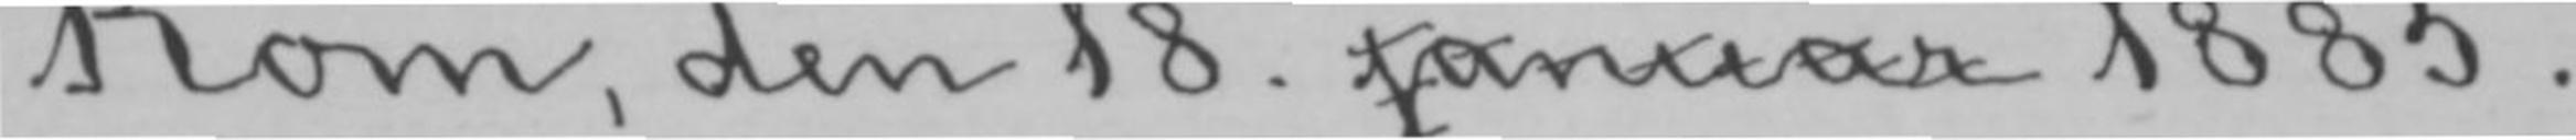Rom, den 18. januar 1885.
